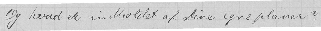Og hvad er indholdet af Dine egne planer?
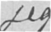jeg
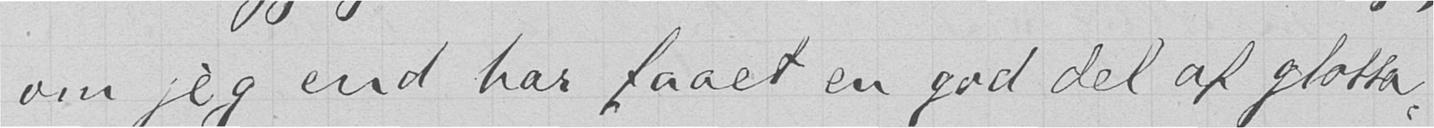om jeg end har faaet en god del af glossa-
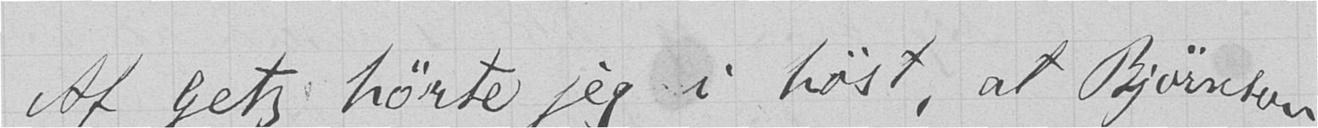Af Getz hørte jeg i høst, at Bjørnson
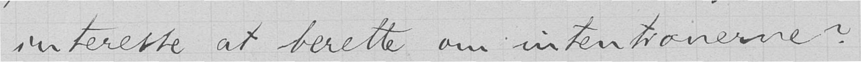interesse at berette om intentionerne?
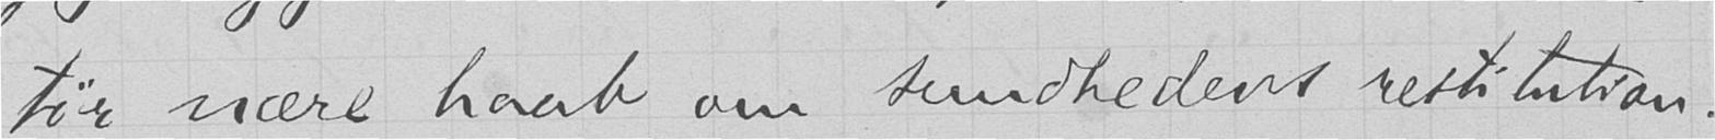tør nære haab om sundhedens restitution.
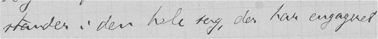stander i den hele sag, der har engageret
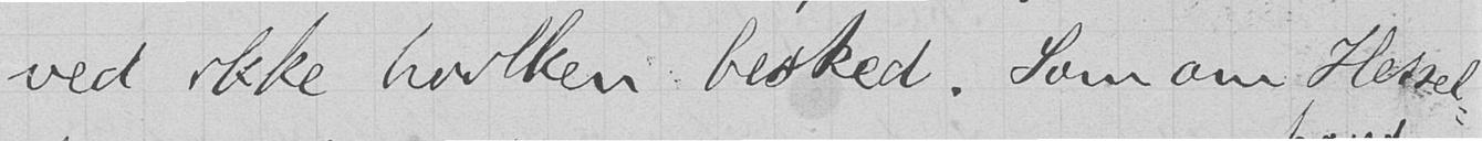ved ikke hvilken besked. Som om Hessel-
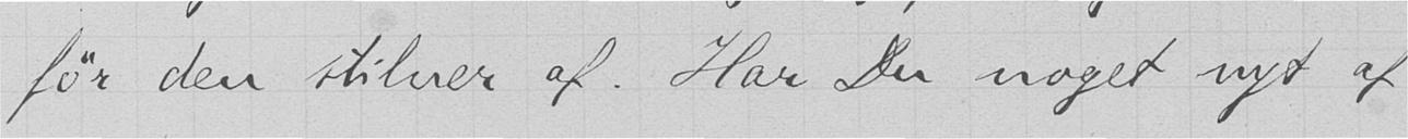før den stilner af. Har Du noget nyt af
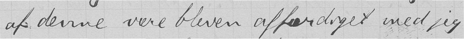af denne være bleven affærdiget med jeg
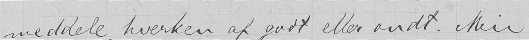meddele, hverken af godt eller ondt. Min
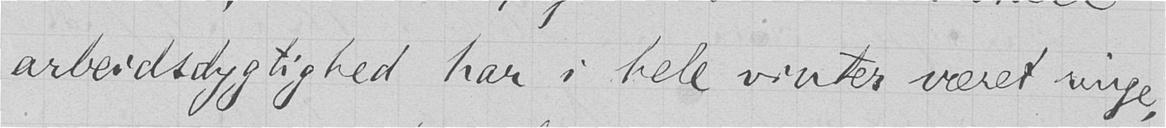arbeidsdygtighed har i hele vinter været rige,
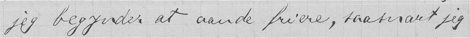jeg begynder at aande friere, saasnart jeg
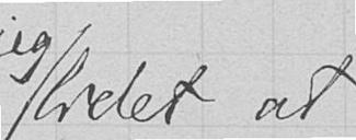lidet at
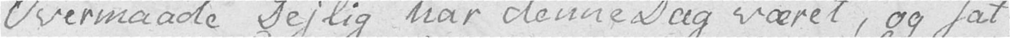Overmaade Dejlig har denne Dag været, og sat
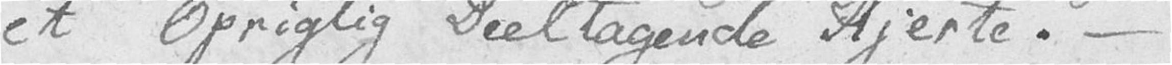et Oprigtig Deeltagende Hjerte. –
